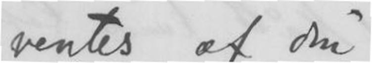ventes af din
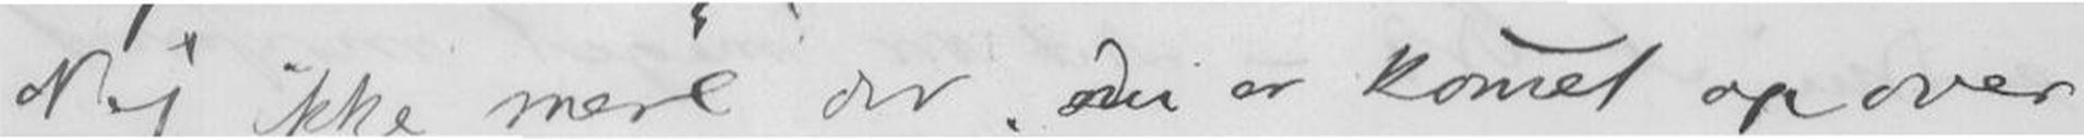Nej ikke mere der, den er komet opover
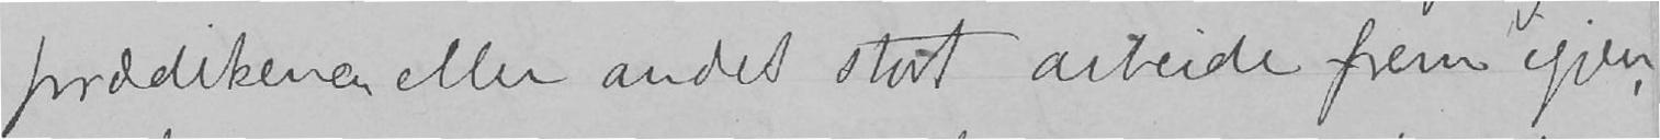prædikenen eller andet stort arbeide frem igjen,
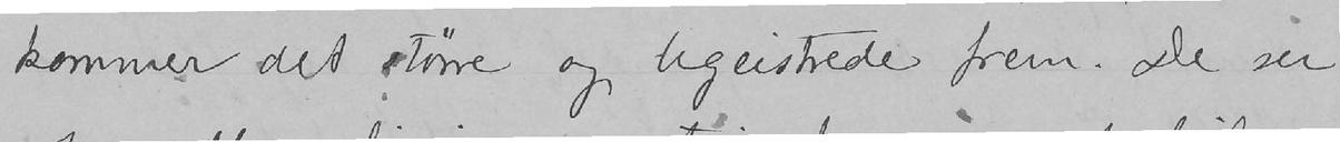kommer det større og begeistrede frem. De ser
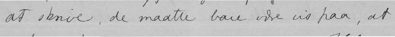at skrive, de maatte bare være vis paa, at
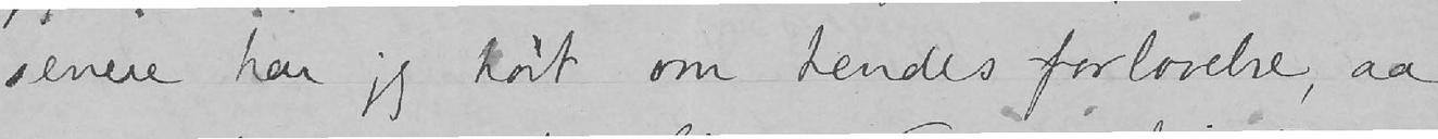senere har jeg hørt om hendes forlovelse, aa
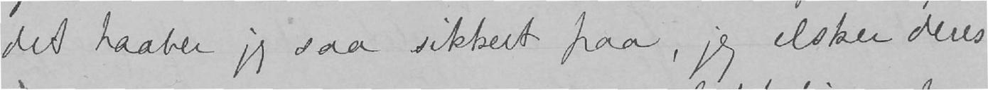det haaber jeg saa sikkert paa, jeg elsker deres
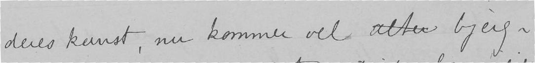deres kunst, nu kommer vel alter bjerg
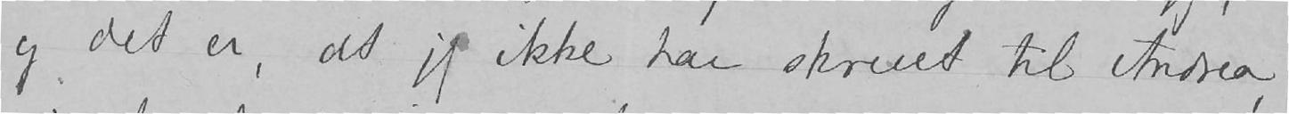og det er, at jeg ikke har skrevet til Andrea,
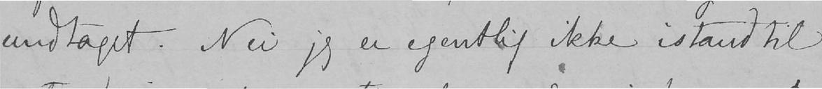undtaget. Nei jeg er egentlig ikke istand til
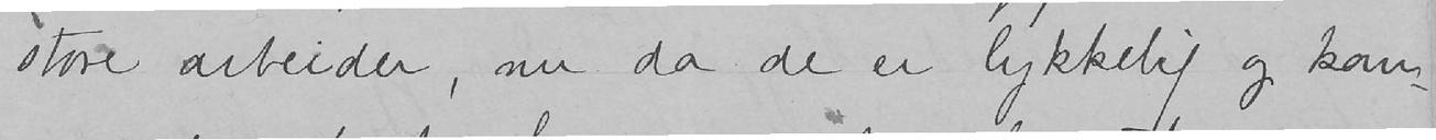store arbeider, nu da de er lykkelig og kom
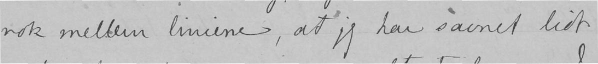nok mellem liniene, at jeg har savnet lidt
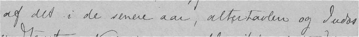af det i de senere aar, altertavlen og Judas
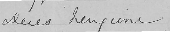Deres hengivne
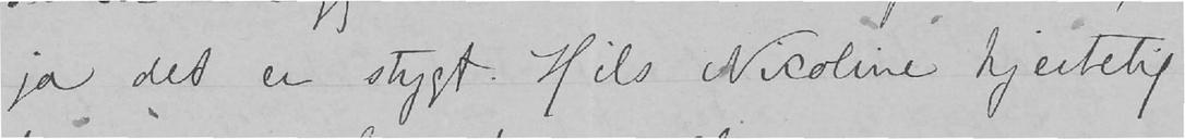ja det er stygt. Hils Nicoline hjertelig
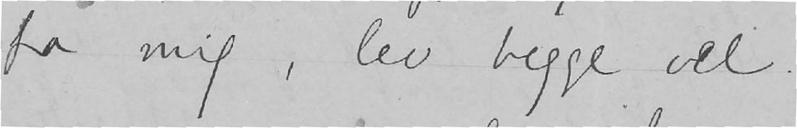fra mig, lev begge vel.
